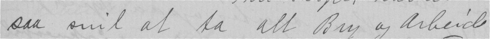saa snil at ta all Bry og arbeide
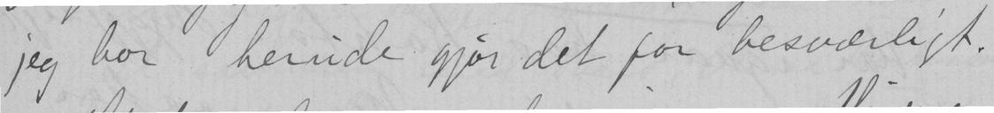jeg bor herude gjør det for besværligt.
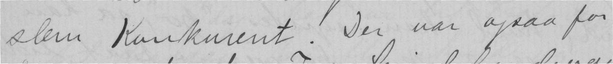slem Konkurrent. Der var ogsaa for
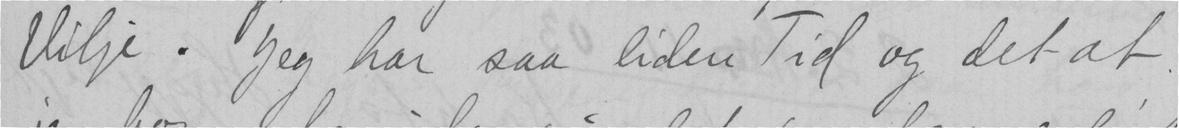vilje. Jeg har saa liden Tid og det at
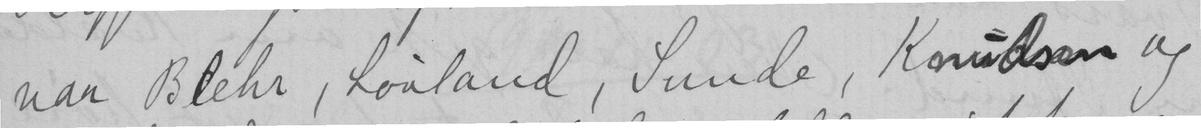var Blehr, Løvland, Sunde, Knudsen og
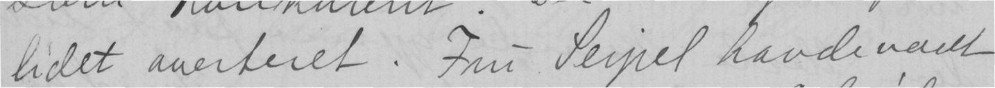lidet averteret. Fru Seipel havde været
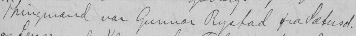thingsmænd var Gunnar Rystad fra Sætusd.
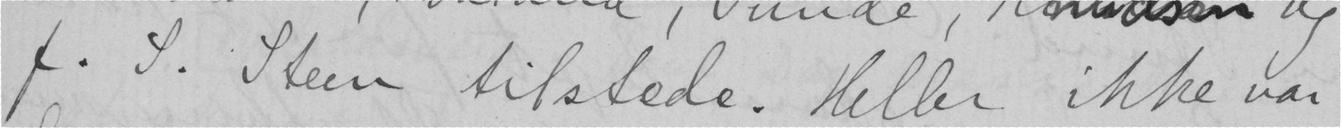f. S. Steen tilstede. Heller ikke var
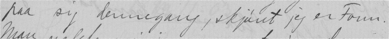paa sig dennegang, skjønt jeg er Form.
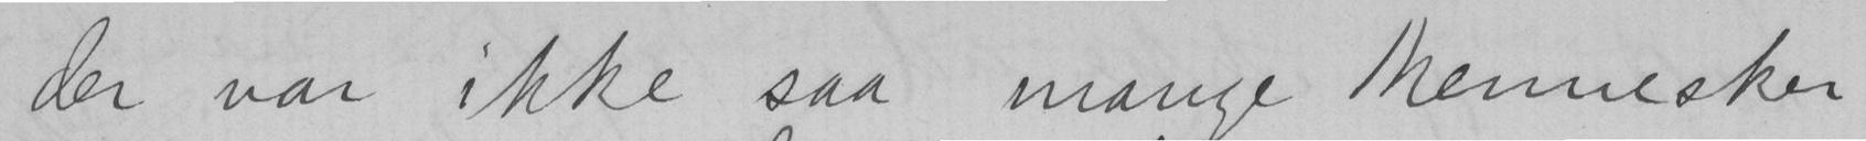der var ikke saa mange mennesker
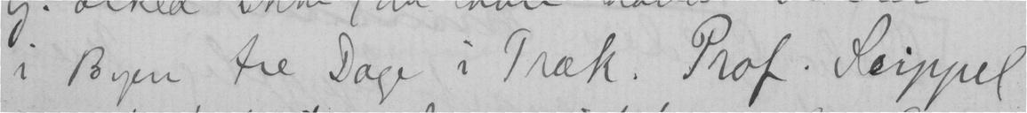i Byen tre Dage i træk. Prof. Seippel
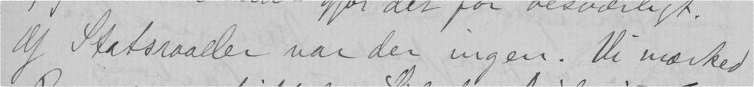Af Statsraader var der ingen. Vi mærked
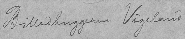Billedhuggeren Vigeland
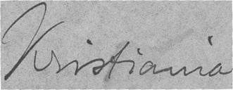Kristiania
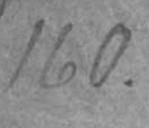160.
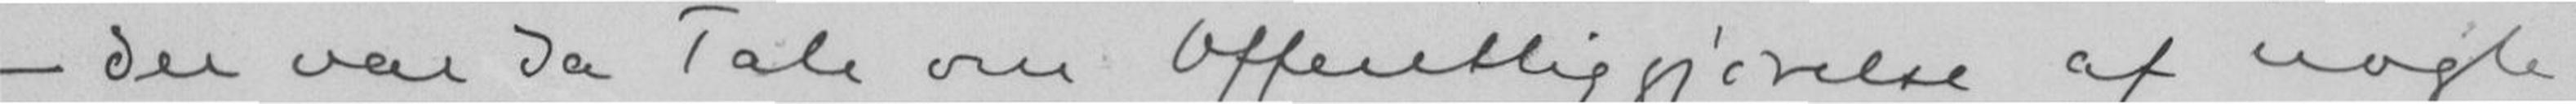- der var da Tale om Offentliggjørelse af nogle
